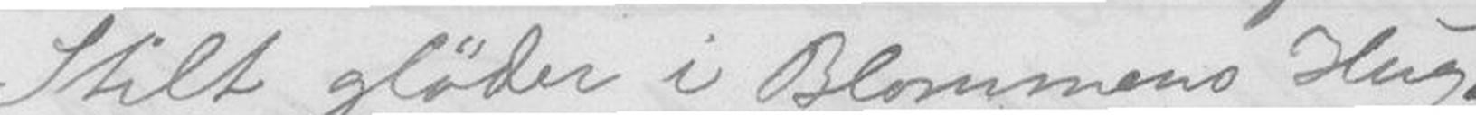Stilt gløder i Blommens Hug.
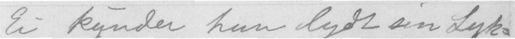Ei kynder hun lydt sin Lyk-
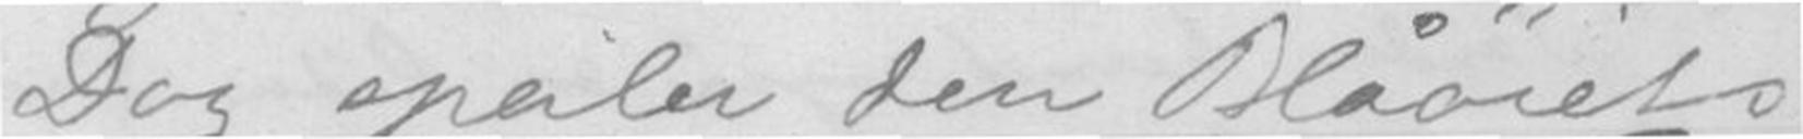Dog speiler den Blåøiets
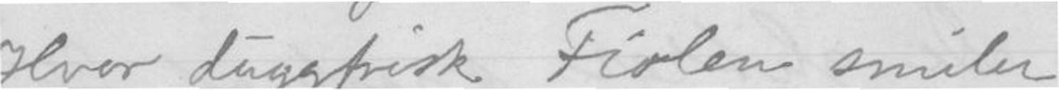Hvor duggfrisk Fiolen smiler
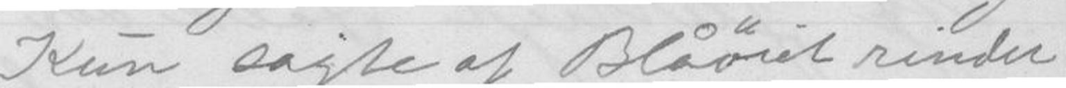Kun sagte af Blåøiet render
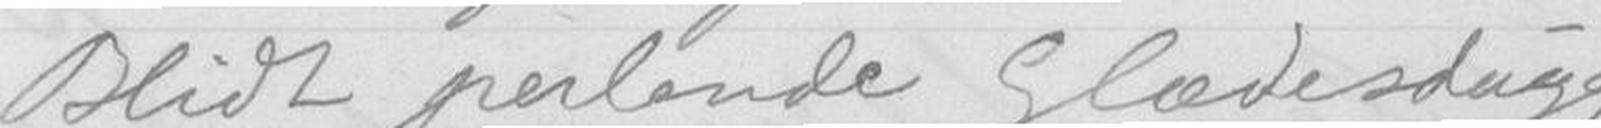Blidt perlende Glædesdugg.
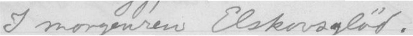I morgenren Elskovsglød.
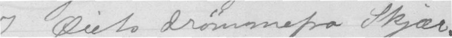I Øiets drømmefra Skjær.
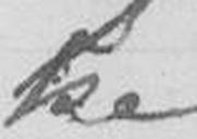ke
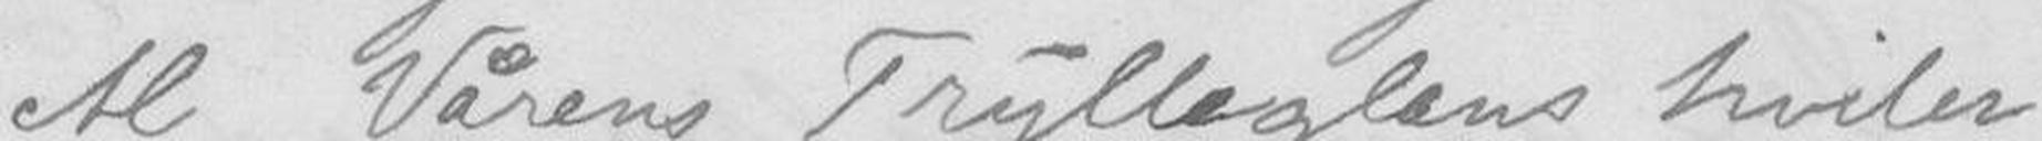Al Vårens Trylleglans hviler
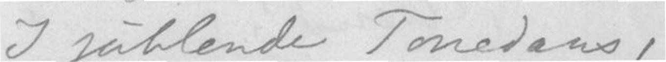I jublende Tonedans,
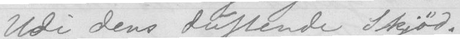Udi dens duftende Skjød.
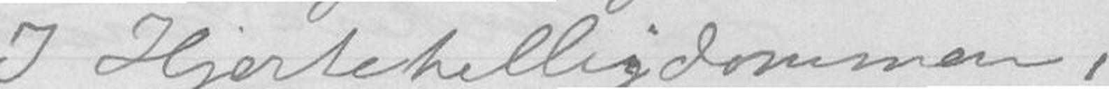I Hjertehelligdommen,
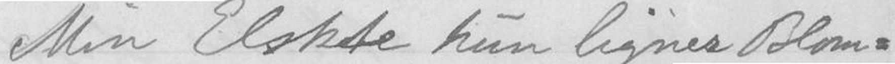Min Elskte kun ligner Blom-
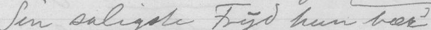Sin saligste Fryd hun bær
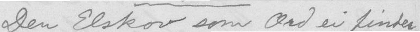Den Elskov som Ord ei finder
